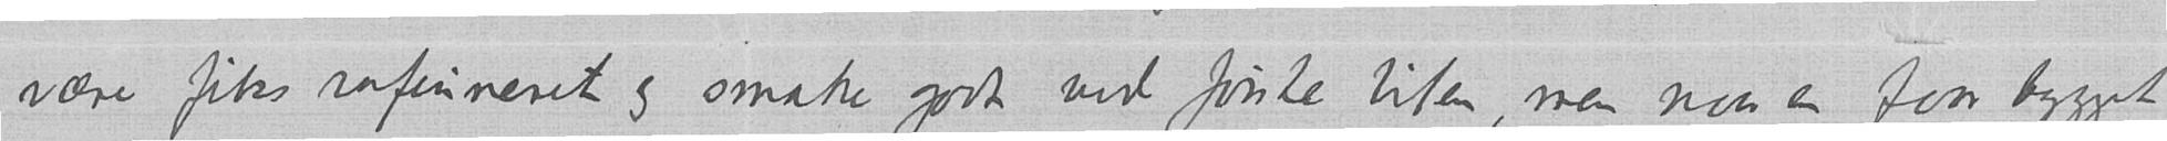være fiks rafinneret og smake godt ved første biten, men naar en faar tygget
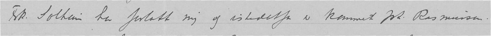Frk. Solheim har forlatt mig og istedatfor ev kommet fru Rasmussen.
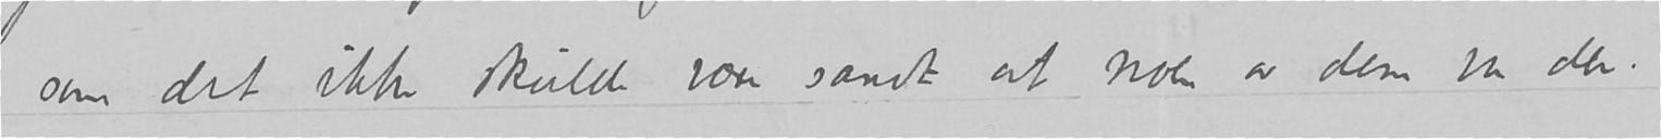som det ikke skulde være sandt at noen av dem var der.
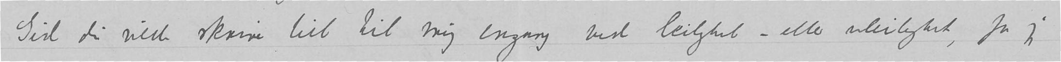Gid du vilde skrive litt til mig engang ved leilighet – eller uleilighet for jeg
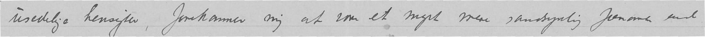usedelige hensigter, forekommer mig at være et meget mere sandsynlig fenomen end
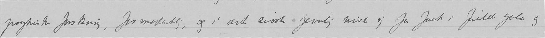psykiske forskning, formodentlig, og i det sisste jevnlig viser sig for folk i fuld gala og
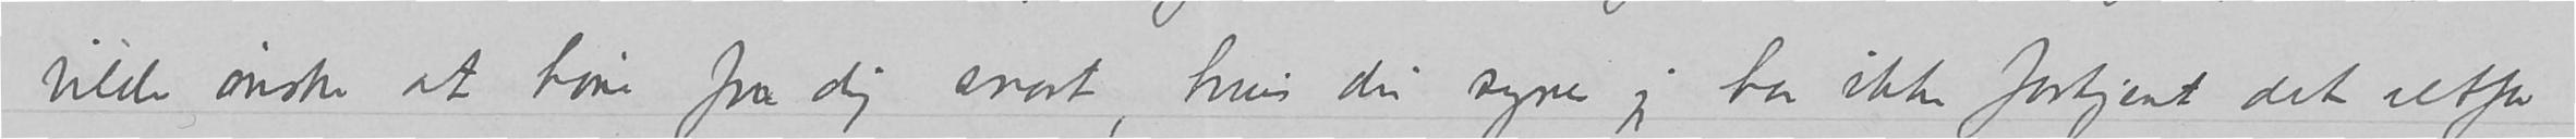vilde ønske at høre fra dig snart, hvis du synes jeg har ikke fortjent det ultfor
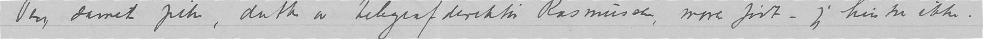Pen dannet pike, datter av telegrafdirektør Rasmussen, more firt – jeg husker ikke.
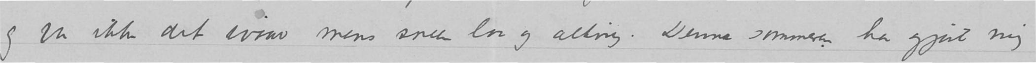og var ikke det ivaar, mens sneen lar og alting. Denne sommeren har gjørt mig
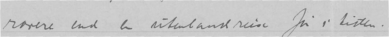ravere end en utenlandreise før i tiden.
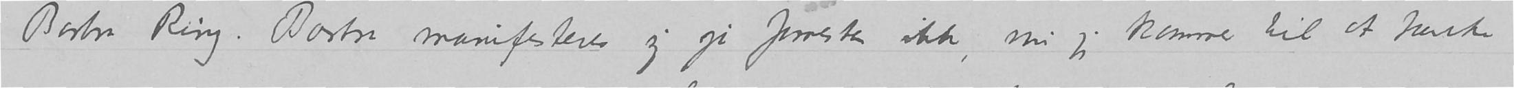Barbra Ring. Barbra manifesteres seg jo forresten ikke, nu jeg kommer til et tænke
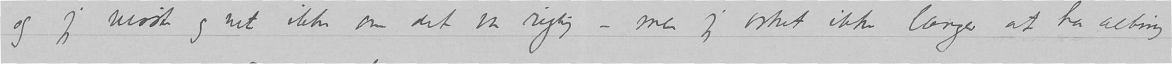og jeg visste og vet ikke om det var rigtig – men jeg orket ikke længer at ha alting
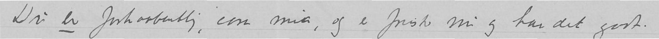Du er forhaabentlig, cora mia, og er frisk nu og har det godt.
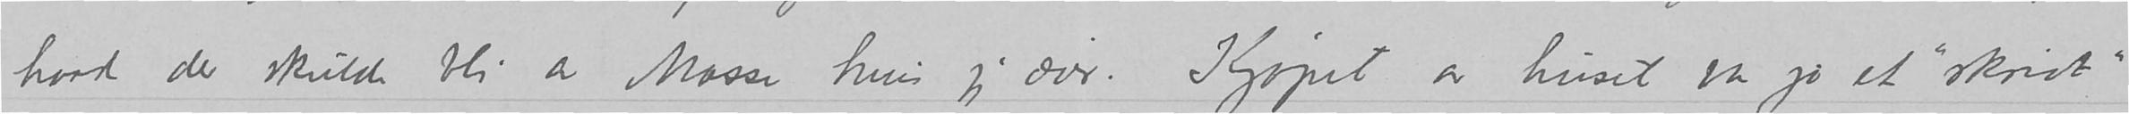hvad der skulde bli av Mosse hvis jeg dør. Kjøpet av huset var jo et «skridt»
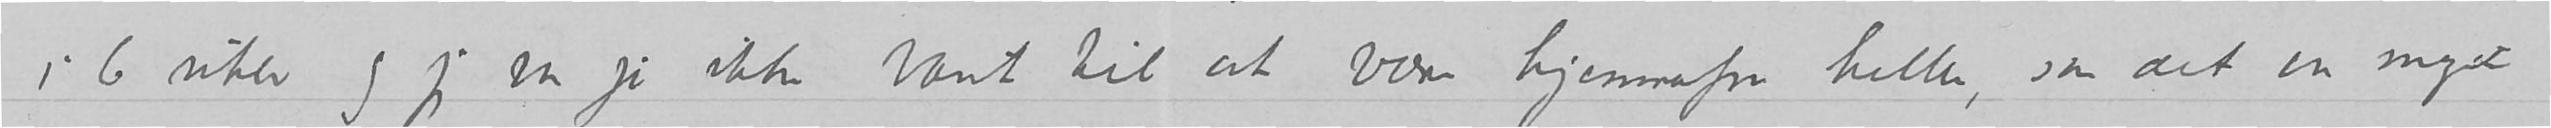i 6 uker og jeg var jo ikke vant til at være hjemmefre heller, saa det er meget
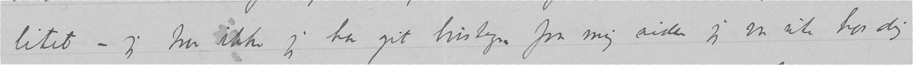litet – jeg tror lkke jeg har git livstegn fra mig siden jeg var ute hos dig
